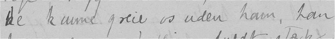de kunne greie os uden ham, han
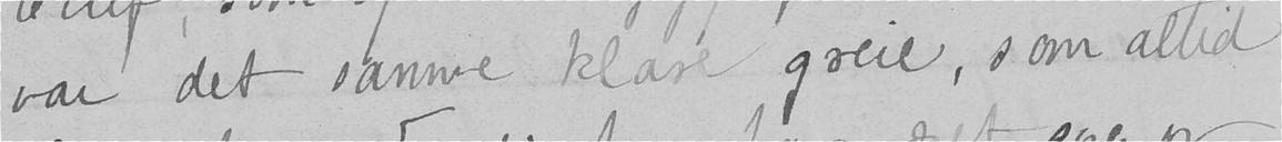var det samme klare greie, som altid
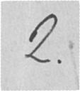2.
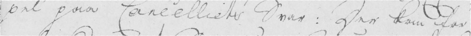pel paa Cancelliets Svar: Der kom for
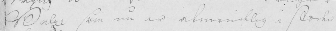Valel som nu er almindelig i stæden
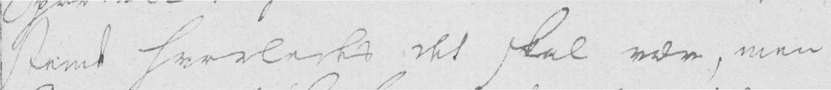stemt hvorledes det skal være, men
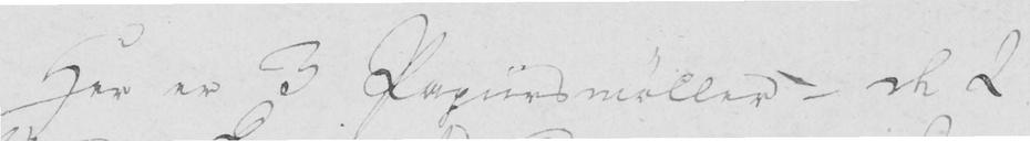Her er 3 Papirsmøller - de 2
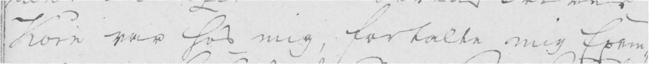Korn var hos mig, fortalte mig Exem-
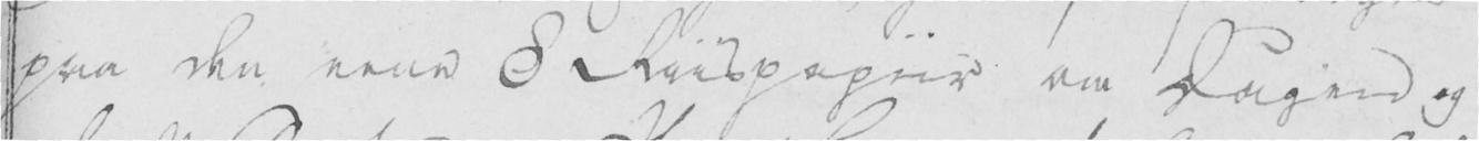paa den eene 8 Riispapiir om Dagen og
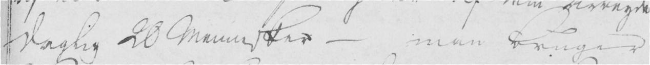daglig 20 Mennisker - man bruger
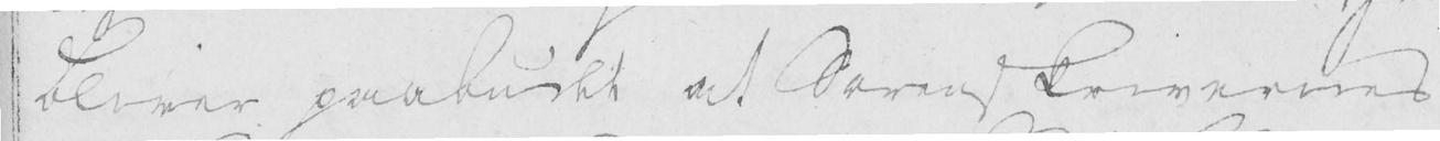bliver paabudet at Sorenskrivernes
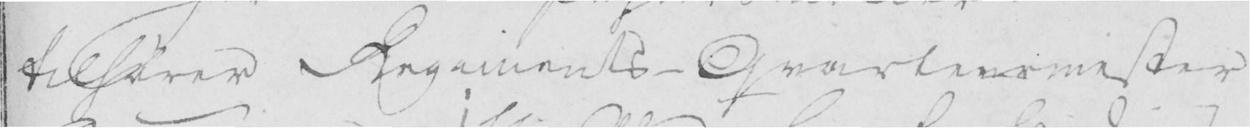tilhører Regiments-Qvartiermester
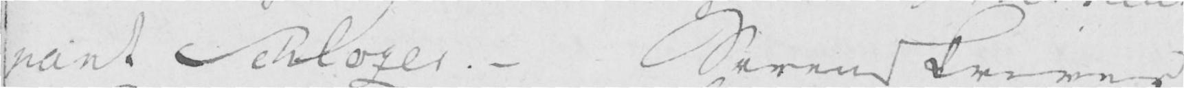nant Schloger. - Sorenskriver
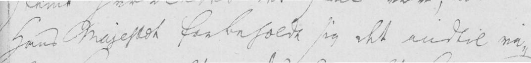Hans Majestæt forbeholde sig det indtil vi-
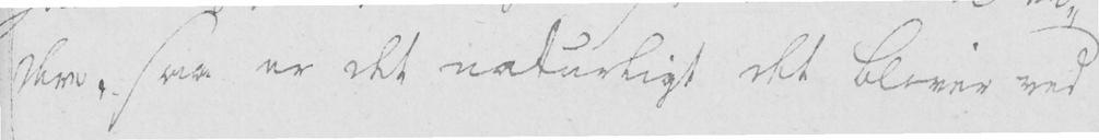dere, saa er det naturligt det bliver ved
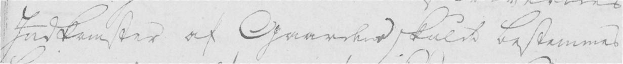Indkomster af Gaarden skulde bestemmes
